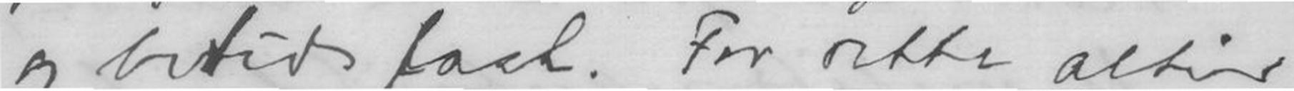og betids fast. For dette altid
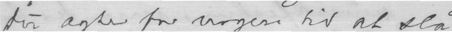du agter for nogen tid at slå
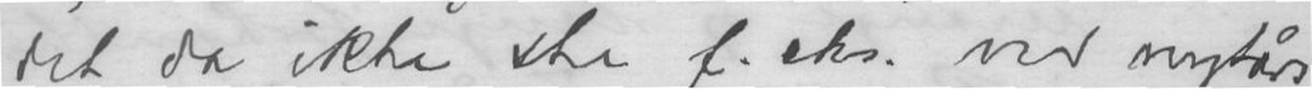det da ikke ske f.eks.ved nytårs
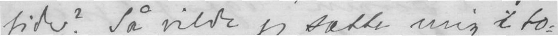tider? Så vilde jeg sætte mig i to-
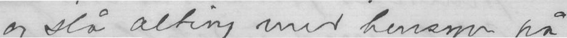og slå alting med hensyn på
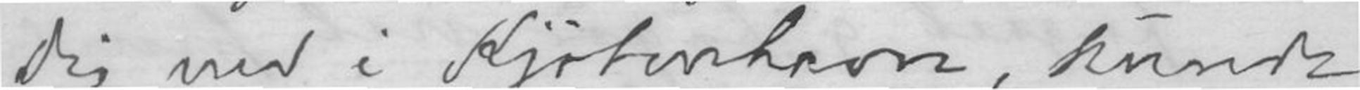dig ned i Kjøbenhavn, kunde
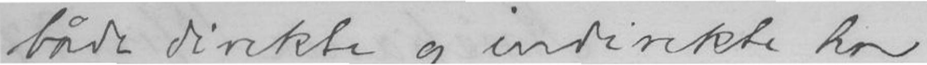både direkte og indirekte har
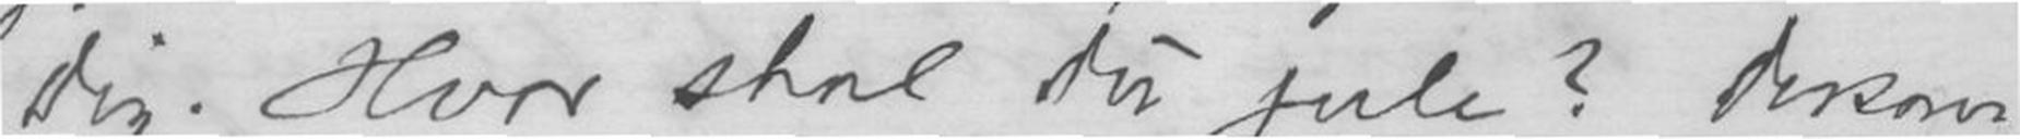dig. Hvor skal du Jule? Dersom
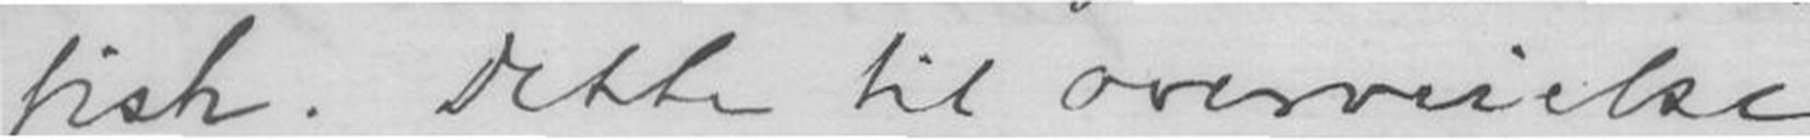tisk. Dette til overveielse.
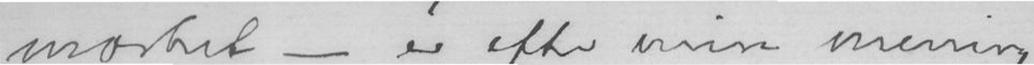mærket - er efter min mening
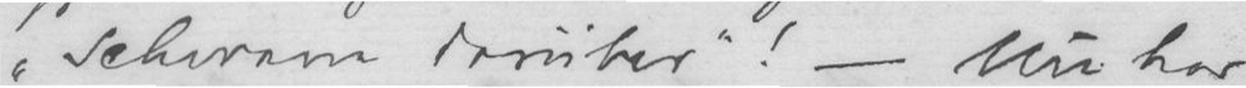"Schwam daruber"!- Nu har
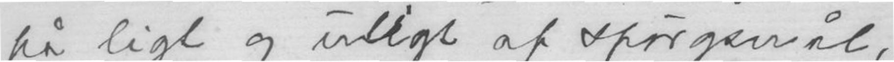på ligt og uligt af spørgsmål,
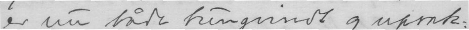er nu både tungvindt og uprak-
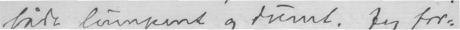både lumpent og dumt. Jeg for-
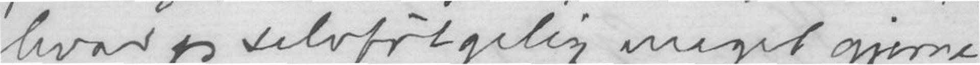hvad jeg selvfølgelig meget gjerne
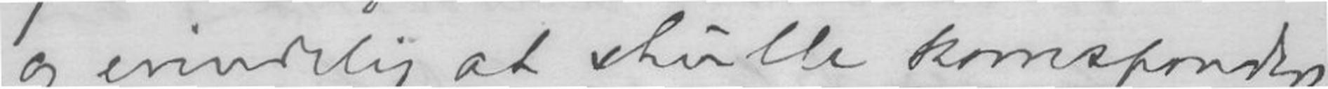og evindelig at skulle korrespondere
